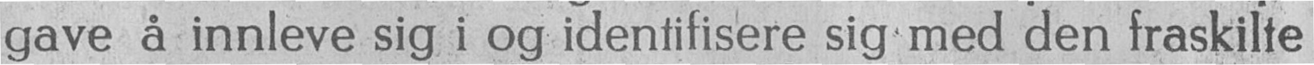gave å innleve sig i og identifisere sig med den fraskilte
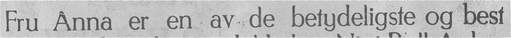Fru Anna er en av de betydeligste og best
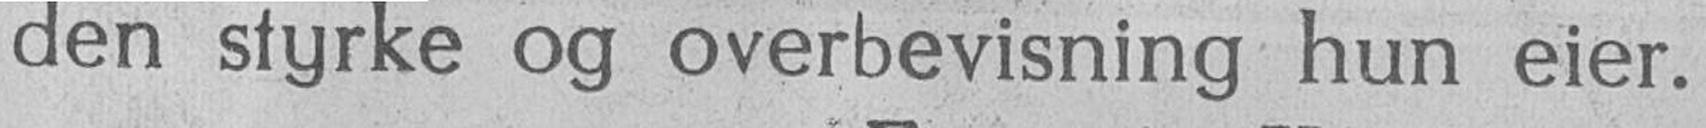den styrke og overbevisning hun eier.
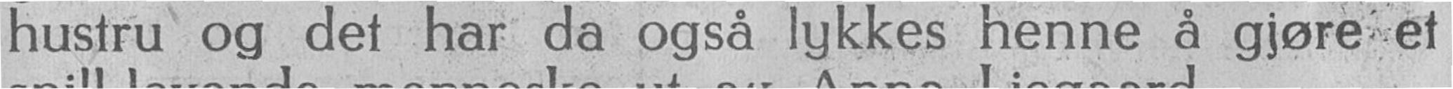hustru og det har da også lykkes henne à gjøre et
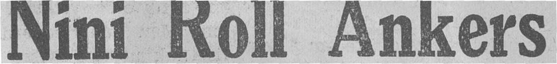Nini Roll Ankers
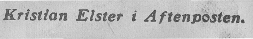Kristian Elster i Aftenposten.
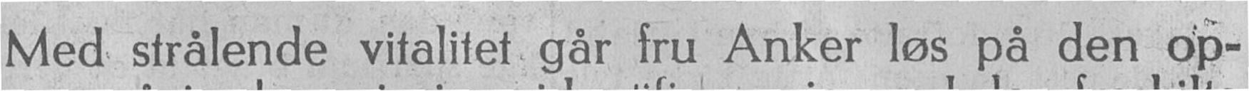Med strålende vitalitet går fru Anker løs på den op-
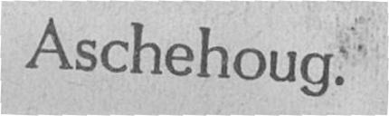Aschehoug.
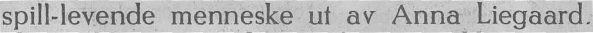spill-levende menneske ut av Anna Liegaard.
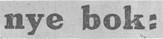nye bok:
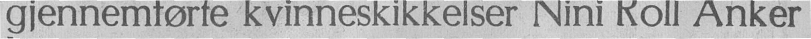gjennemførte kvinneskikkelser Nini Roll Anker
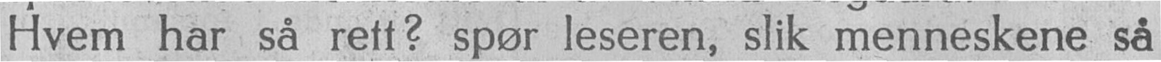Hvem har så rett? spør leseren, slik menneskene så
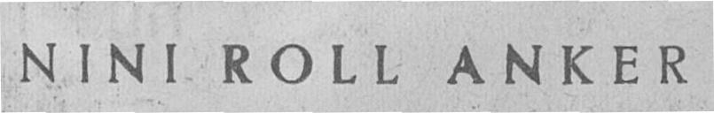NINI ROLL ANKER
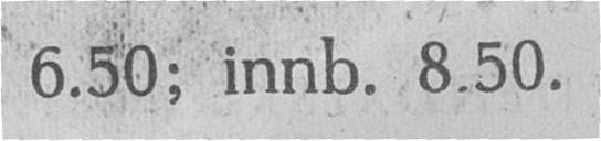6.50; innb. 8.50.
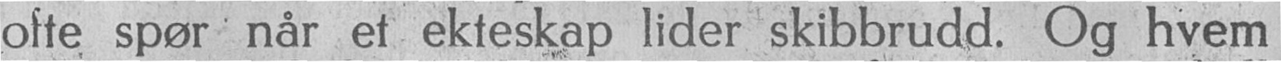ofte spør når et ekteskap lider skibbrudd. Og hvem
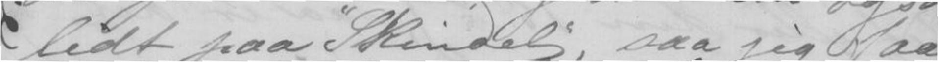lidt paa Skindet", saa jeg faa
